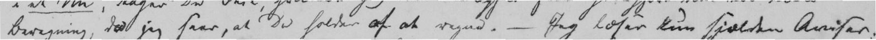Beregning, da jeg seer, at De holder af at regne. - Jeg læser kun sjælden Aviser;
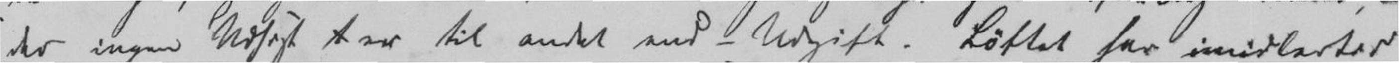de ingen Udsigt er til andet end - Udgift. Løftet har imidlertid
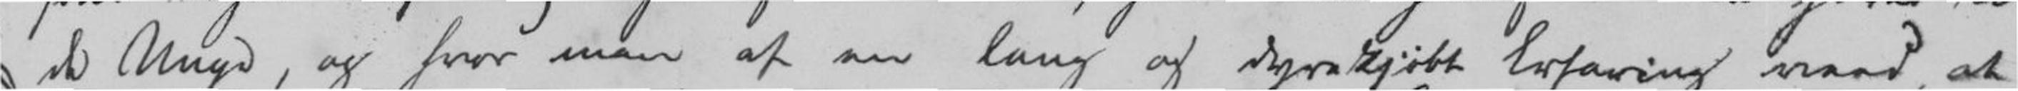de Unge, og hvor man af en lang og dyrekjøbt Erfaring veed, at
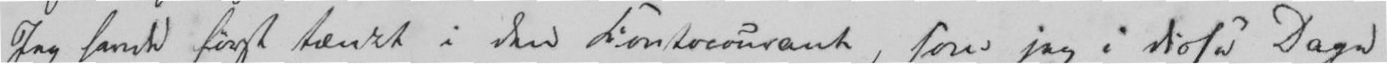Jeg havde først tænkt i den Contocourant, som jeg i disse Dage
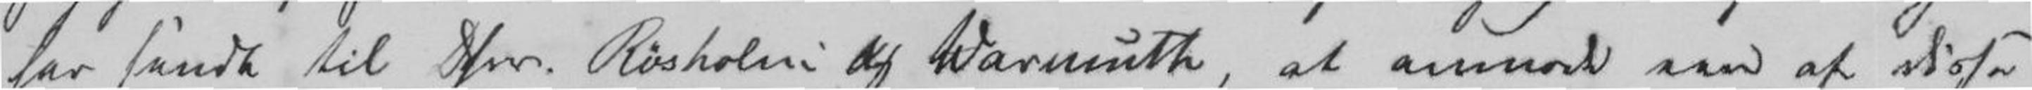har sendt til Dhrr. Røsholm og Warmuth, at anmode een af disse
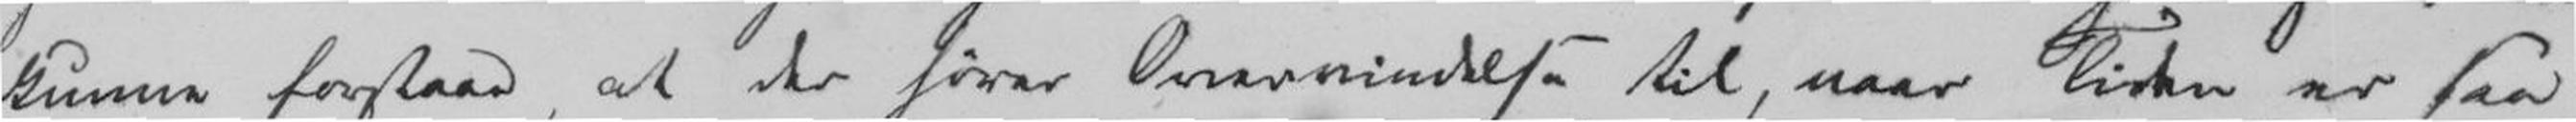kunne forstaae, at der hører Overvindelse til, naar Tiden er saa
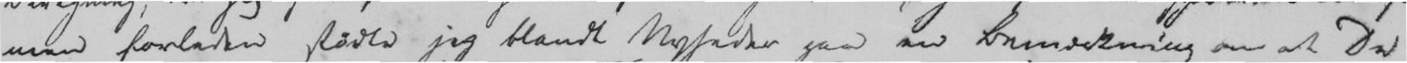men forleden stødte jeg blandt Nyheder paa en Bemærkning om at De
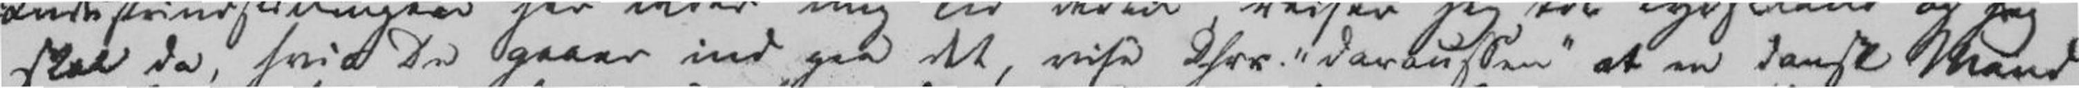skal da, hvis De gaaer ind paa det, reise Dhrr. "darausken" at en dansk Mand
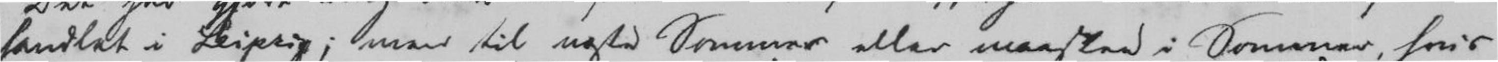handlet i Leipzig; men til næste Sommer eller maaskee i Sommer, hvis
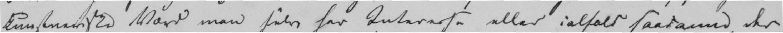Kunstnerske Værd man selv har Interesse eller ialtfald saadanne, der
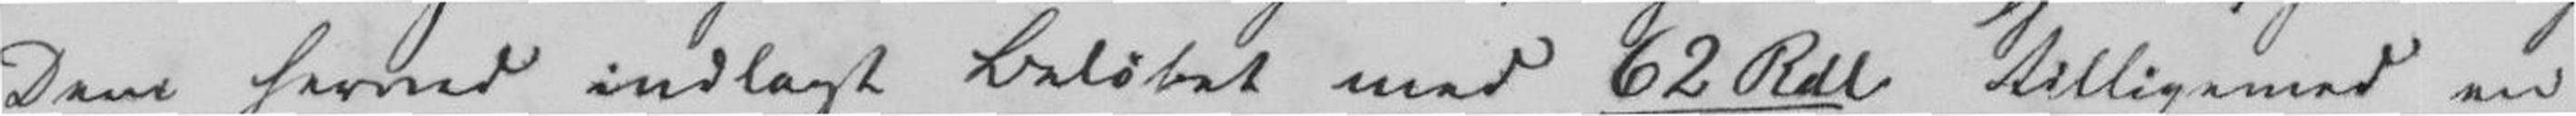Dem herved indlagt Beløbet med 62 Rdl tilligemed en
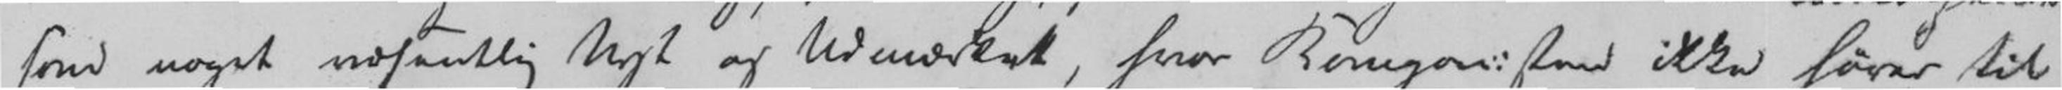som noget væsentlig Nyt og Udmærket, hvor Komponisten ikke hører til
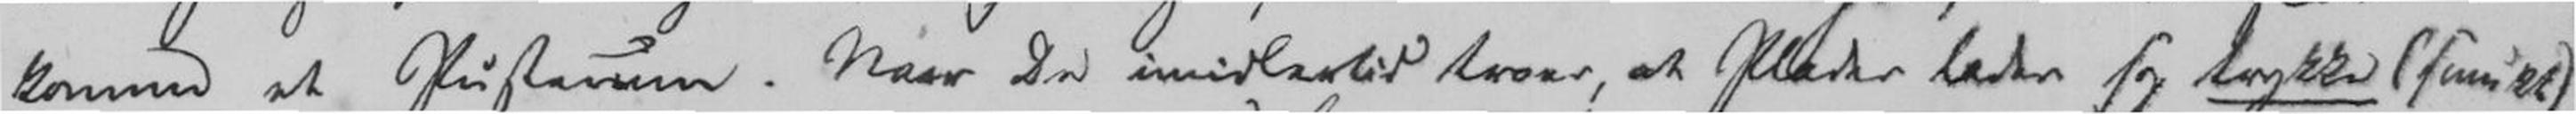komme et Pusterum. Naar De imidlertid troer, at Plader lader sig trykke (smukt)
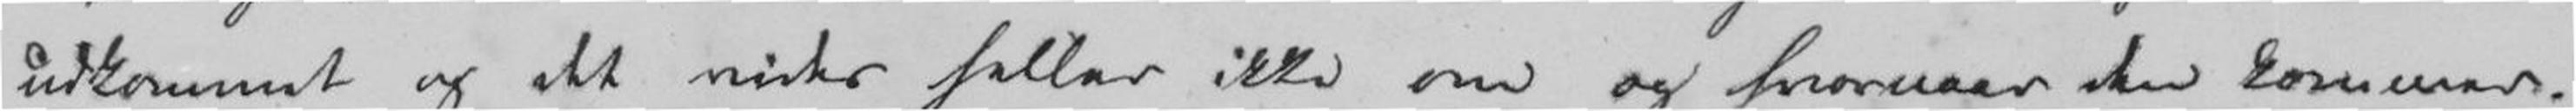udkommet og det vides heller ikke om og hvornaar den kommer. -
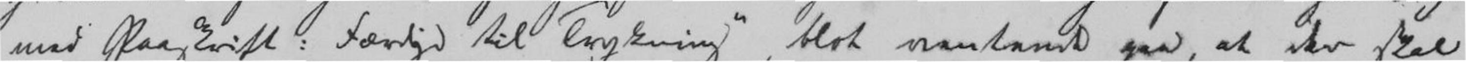med Paaskrift: Færdige til Trykning", blot væntende paa, at der skal
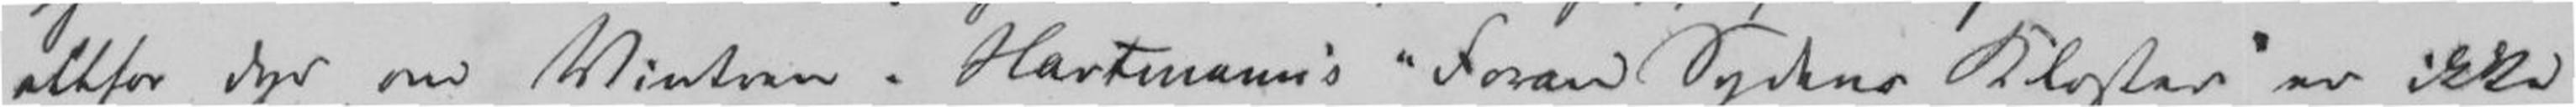altfor dyr om Vinteren. Hartmanns "Foran Sydens Kloster" er ikke
